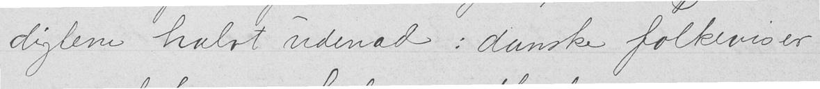digtene halvt udenad: danske folkeviser
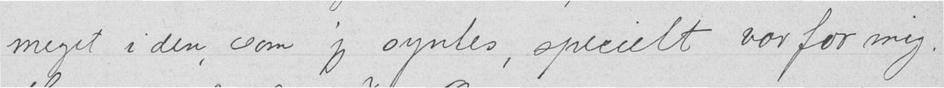meget i den, som jeg syntes, specielt var for mig.
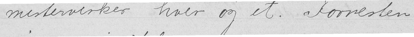mesterverker hver og et. Forresten
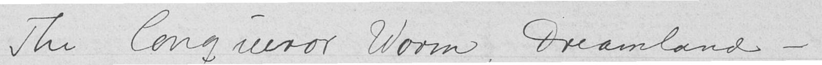The Conqueror Worm, Dreamland -
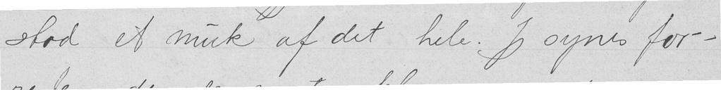stod et muk af det hele. Jeg synes for-
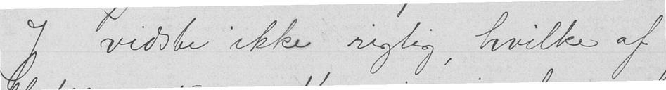Jeg vidste ikke rigtig, hvilke af
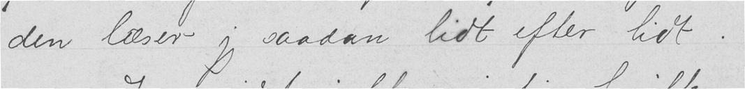den læser jeg saadan lidt efter lidt.
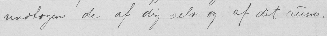undtagen de af dig selv og af dit rum.
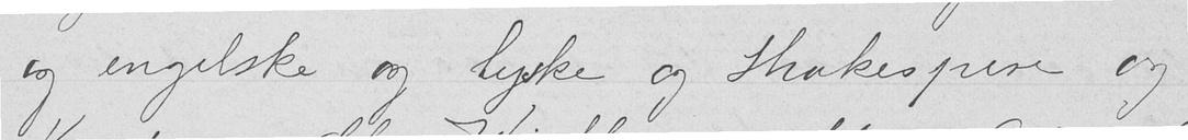og engelske og tyske og Shakespere og
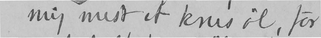mig mest et krus øl, før
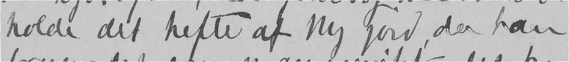holde det hefte af ny jord, da han
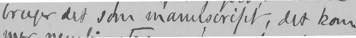bruger det som manuscript, det kom-
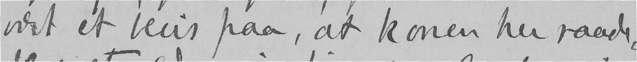vært et bevis paa, at konen her raade-
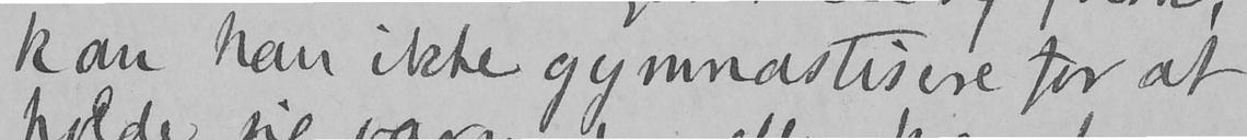kan han ikke gymnastisere for at
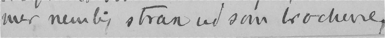mer nemlig strax ud som brochure.
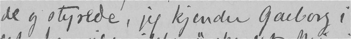de og styrede, jeg kjender Garborg i
